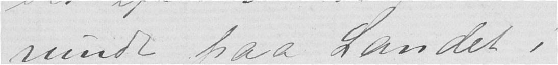rundt paa Landet i
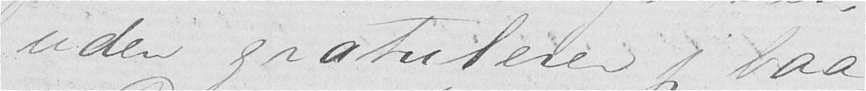uden gratulerer jeg baa
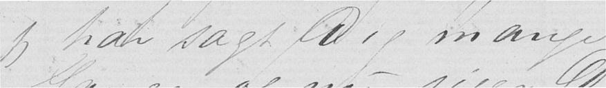jeg har sagt Dig mange
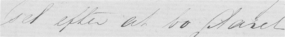sel efter at bo Aaret
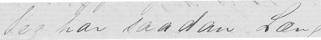Jeg har saadan Læng-
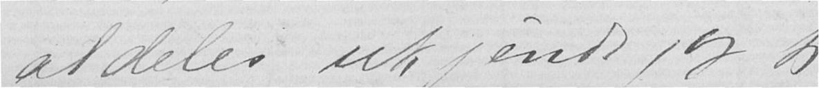aldeles ukjendt, og jeg
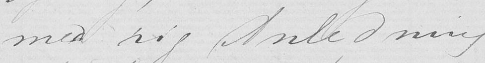med rig Anledning
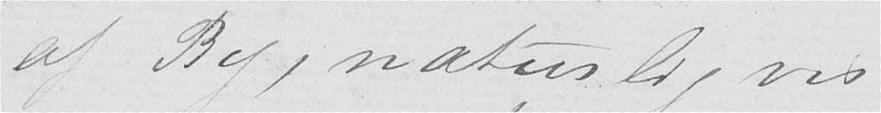af By, naturlig vis
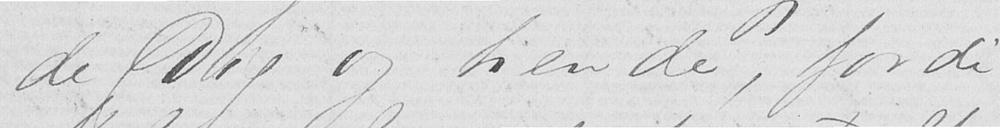de Dig og hende, fordi
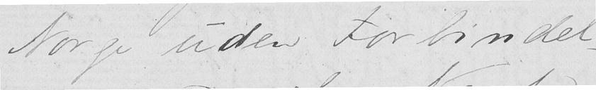Norge uden Forbindel-
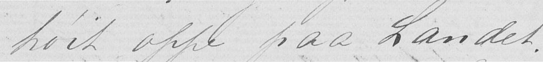høit oppe paa Landet.
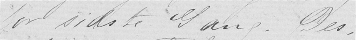for sidste Gang! Des-
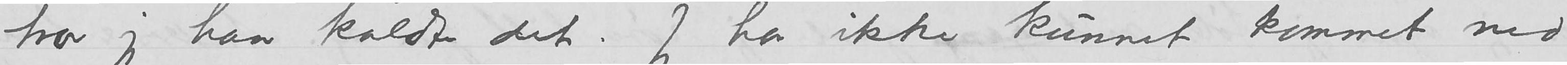tror jeg han kaldte det. Jeg har ikke kunnet kommet
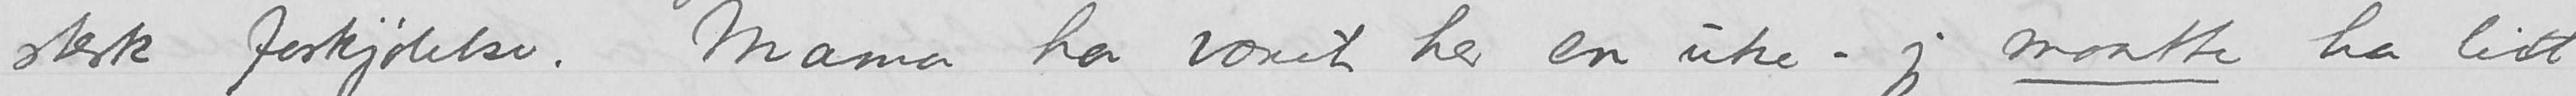sterk forkjølelse. Mama har været her en uke - jeg maatte ha litt
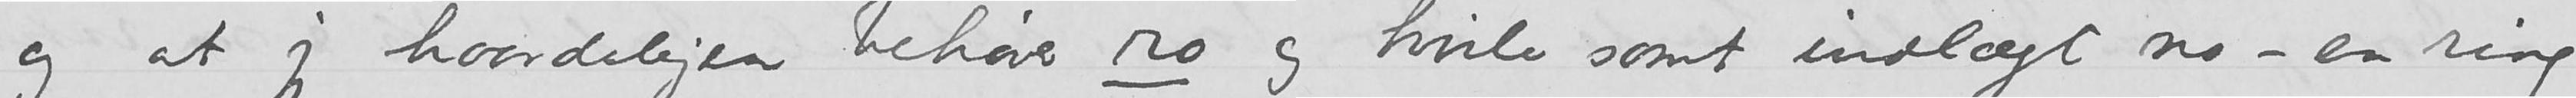og at jeg haardeligen behøver ro og hvile samt indlagt no - en ring
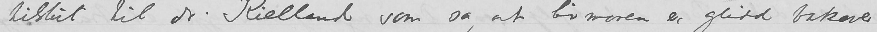tilslut til dr. Kielland som sa at livmoren er glidd bakover
